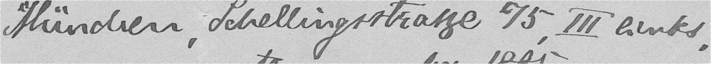München, Schellingsstrasse 75, III cinks,
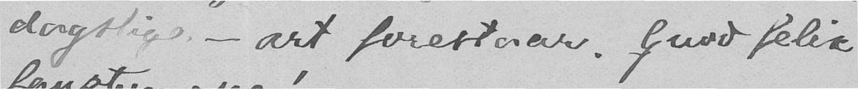dagslige – art forestaar. Quod felix
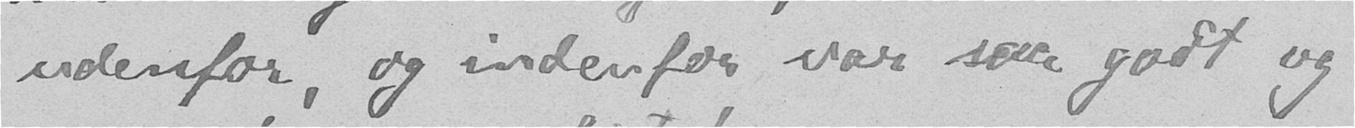udenfor, og indenfor var saa godt og
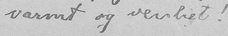varmt og venligt!
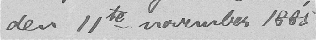den 11te november 1885.
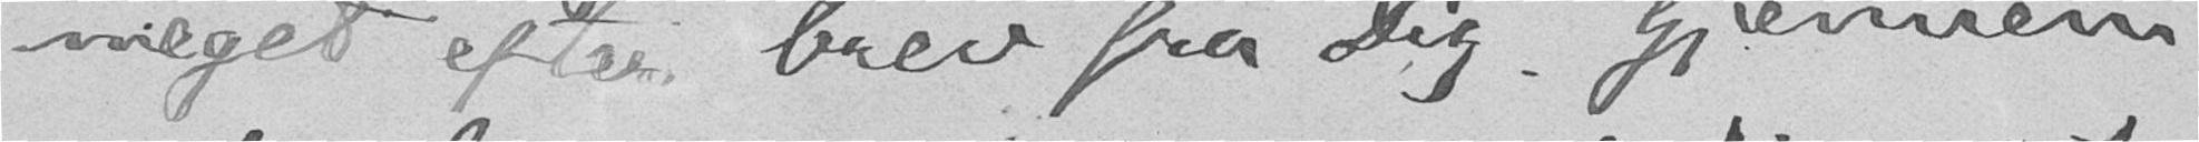meget efter brev fra Dig. Gjennem
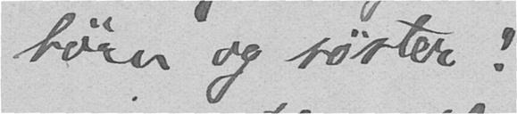børn og søster!
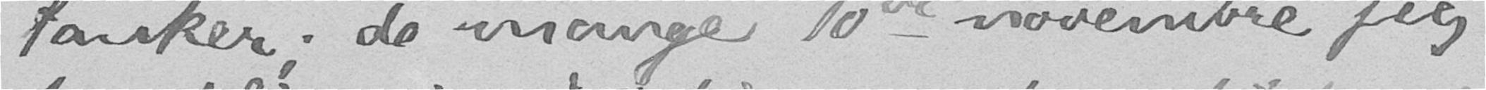tanker; de mange 10de novembre jeg
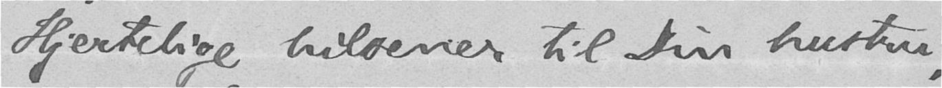Hjertelige hilsener til Din hustru,
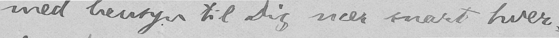med hensyn til Dig nær snart hver-
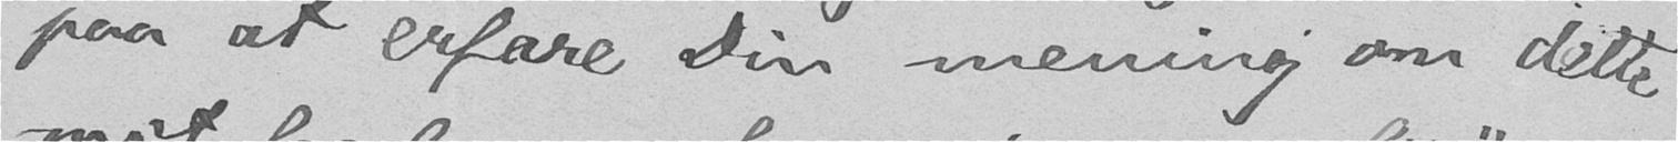paa at erfare Din mening om dette
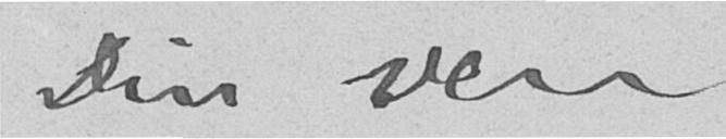Din ven
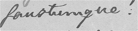faustumque!
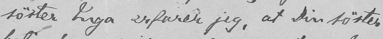søster Inga erfarer jeg, at Din søster
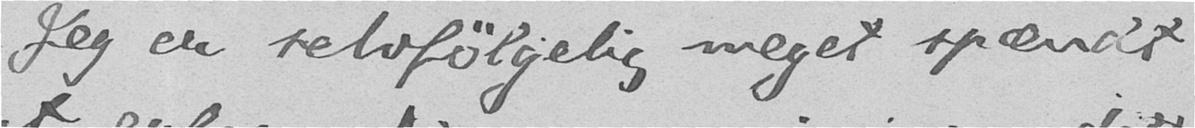Jeg er selvfølgelig meget spændt
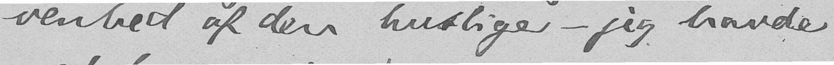venhed af den huslige – jeg havde
